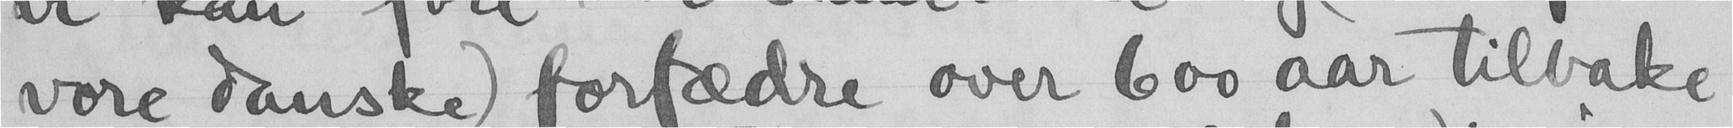vore danske) forfædre over 600 aar tilbake,
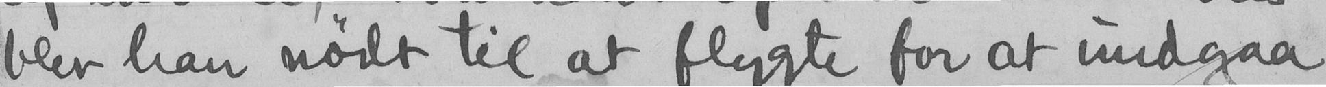blev han nodt til at flygte for at undgaa
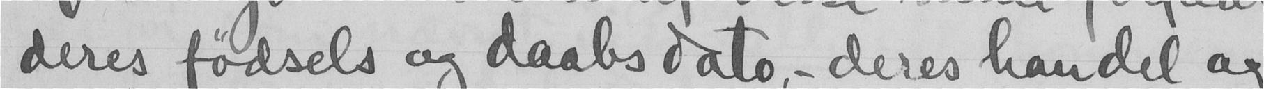deres fødsels og daabs dato, - deres handel og
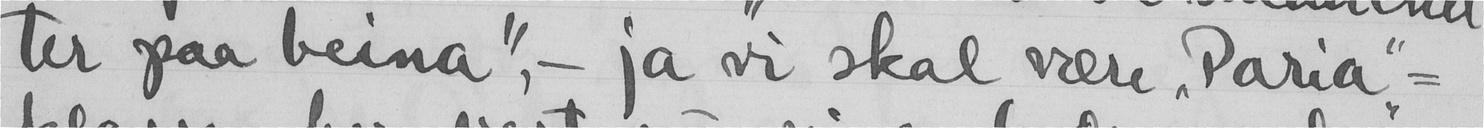ter paa beina", - ja vi skal være "Paria"-
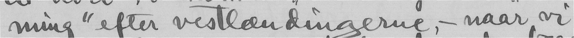ming" efter vestlændingerne, - naar vi
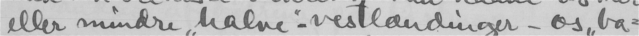eller mindre "halve"-vestlændinger - os "ba-
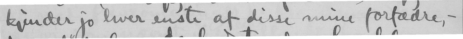kjender jo hver enste af disse mine forfædre,-
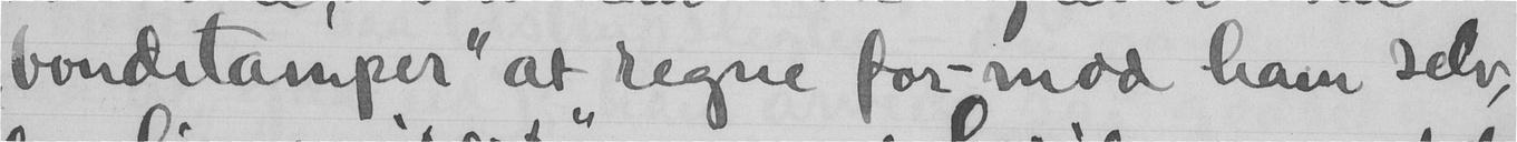"bondetamper" at regne for - mod ham selv,
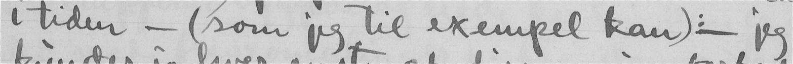i tiden - (som jeg til exempel kan): - jeg
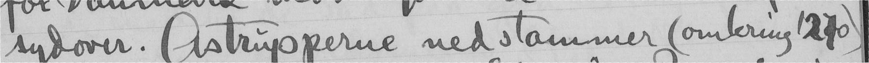sydover. Astrupperne nedstammer (omkrin 1270)
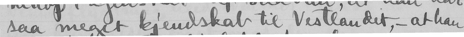saa meget kjendskab til Vestlandet, - at han
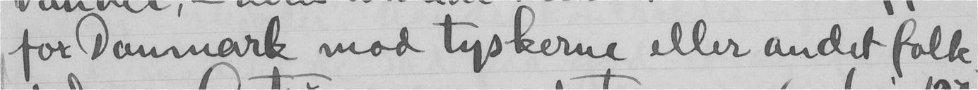for danmark mod tyskerne eller andet folk
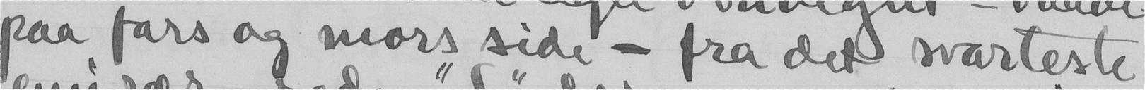paa fars og mors side - fra det svarteste
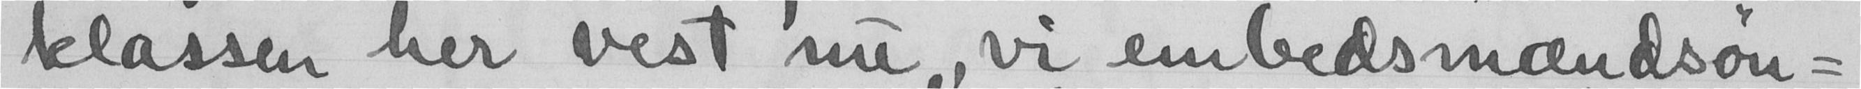klassen her best nu, vi enbedsmændsøn-
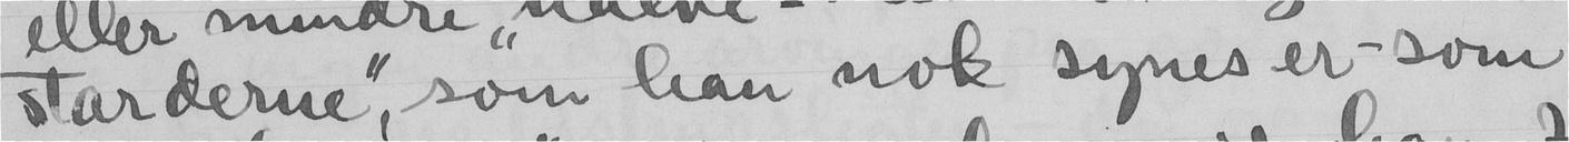starderne", som han nok synes er - som
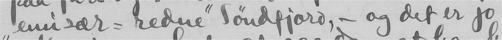"emisær-redne" Søndfjord, - og det er jo
